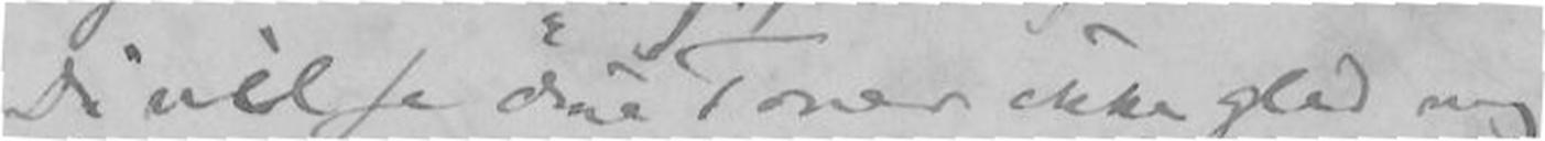Du vil se dine Toner ikke gled mig
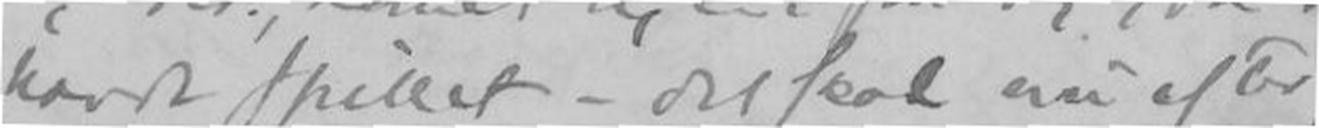havde spillet - det skal nu efter
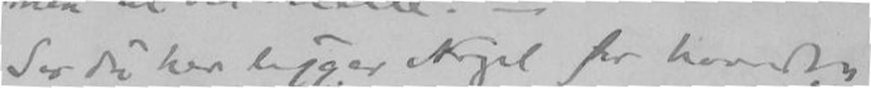Ser du her ligger Noget for hovedlig
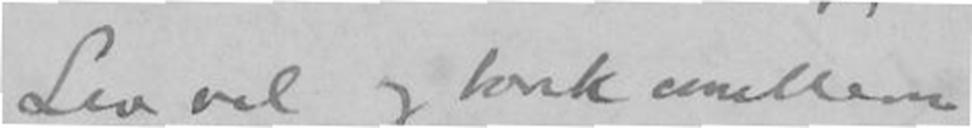Lev vel og tænk imellem
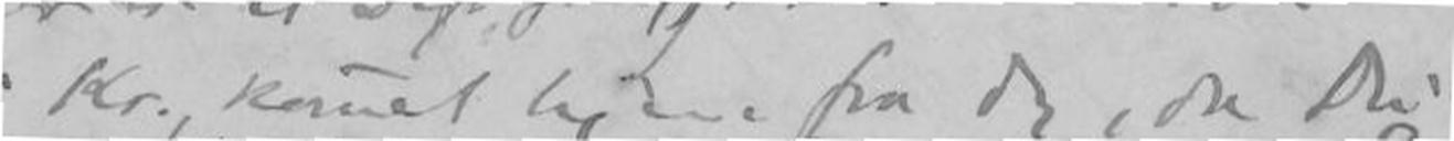i Kr., komet lige fra Dig, de Du
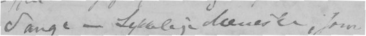Sange - Lykkelig Menneske, som
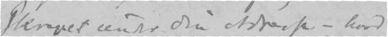skrevet under din Adresse - hvad
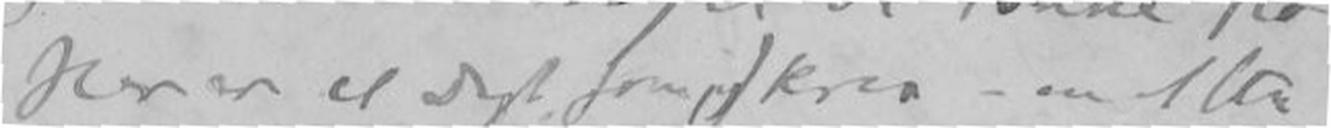Her er et Digt som jeg skrev - en Aften
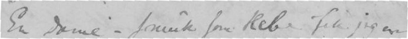En Dame - smuk som Rebe fik jeg en
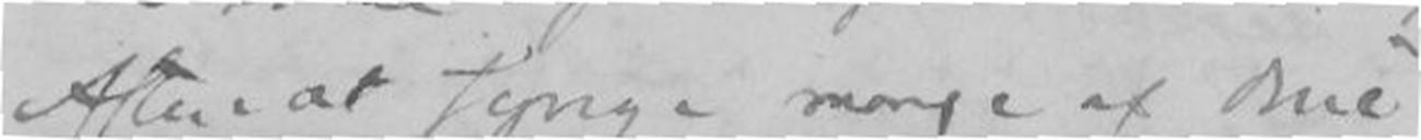Aften at synge mange af dine
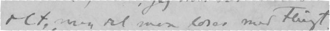alt, men det maa læses med Flugt
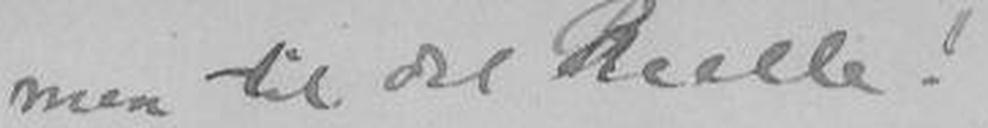men til det Reelle!
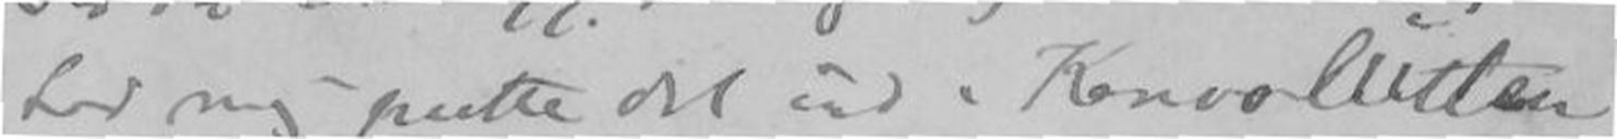Lod mig putte det ind i Konvolutten
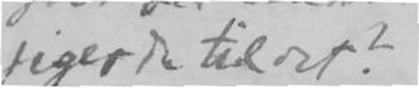siger du til det?
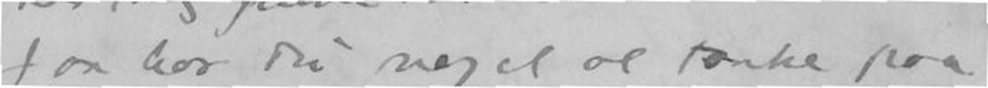saa har du noget at tænke paa
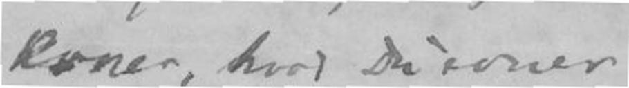Raner, hvad Du evner
